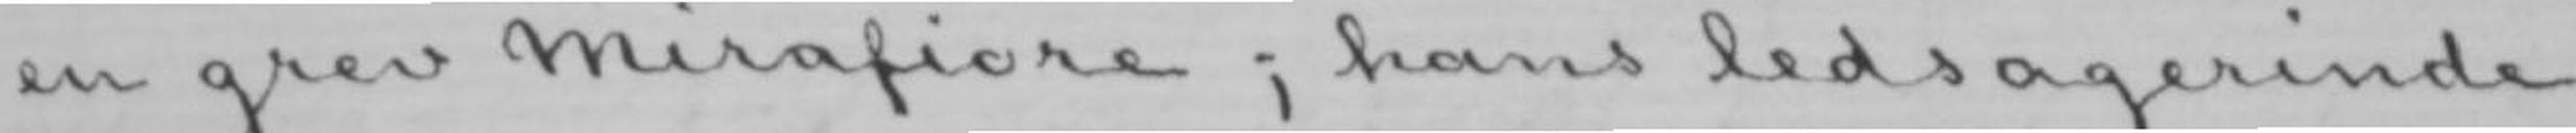en grev Mirafiore; hans ledsagerinde
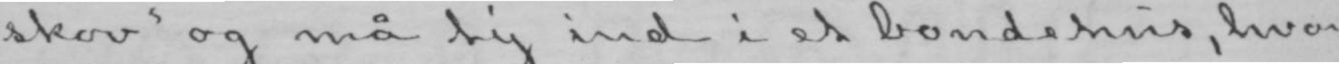skov» og må ty ind i et bondehus, hvor
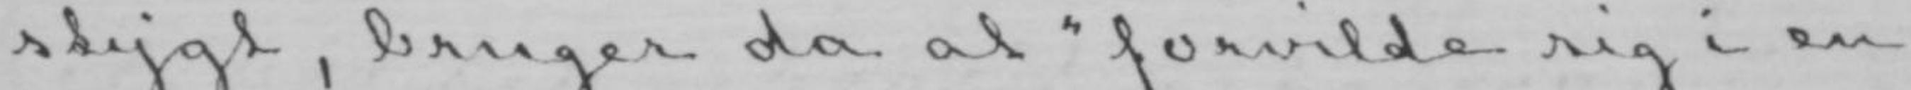stygt, bruger da at «forvilde sig i en
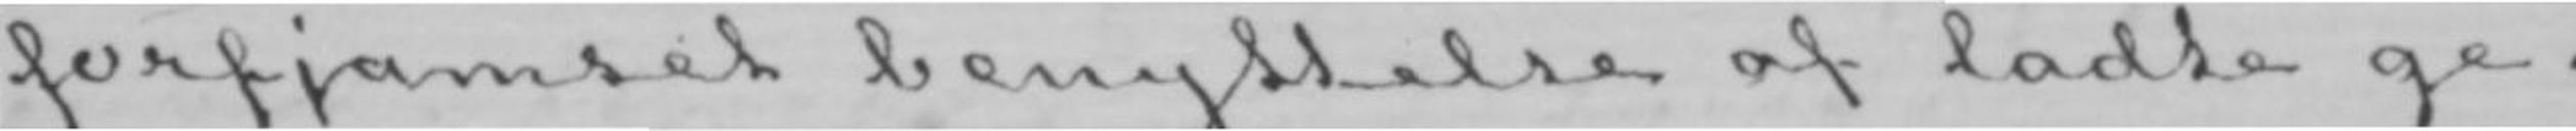forfjamset benyttelse af ladte ge-
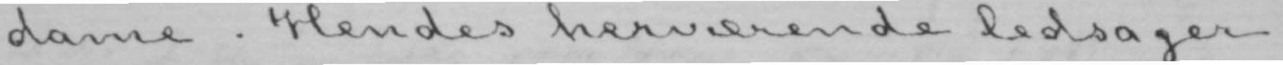dame. Hendes herværende ledsager
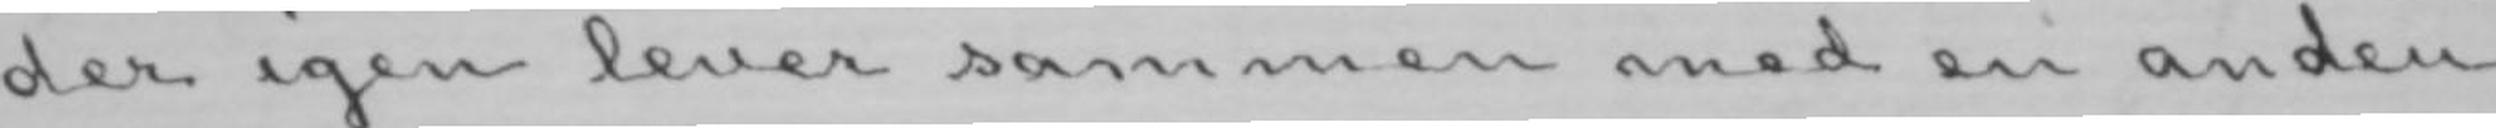der igen lever sammen med en anden
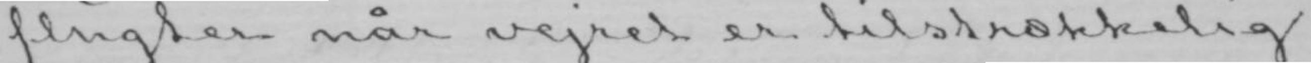flugter når vejret er tilstrækkelig
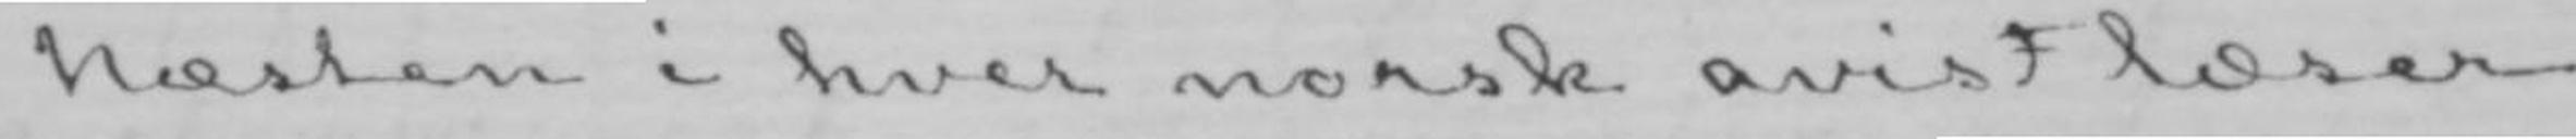Næsten i hver norsk avis  læser
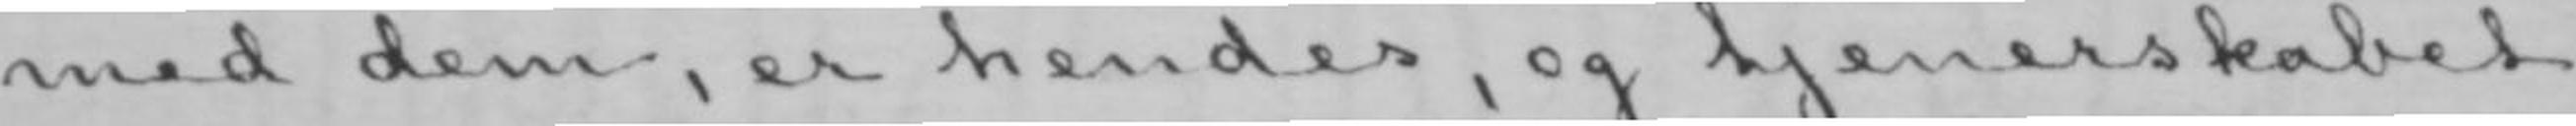med dem, er hendes, og tjenerskabet
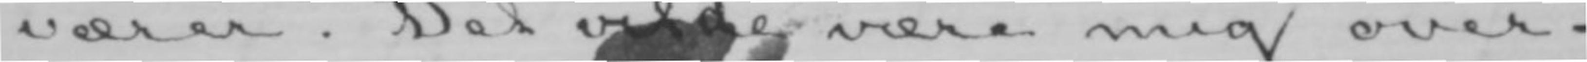værer. Det vilde være mig over-
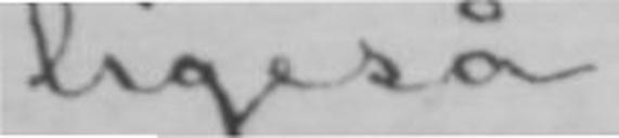ligeså.
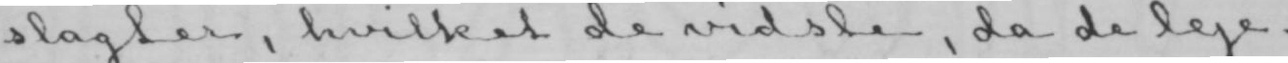slagter, hvilket de vidste, da de leje-
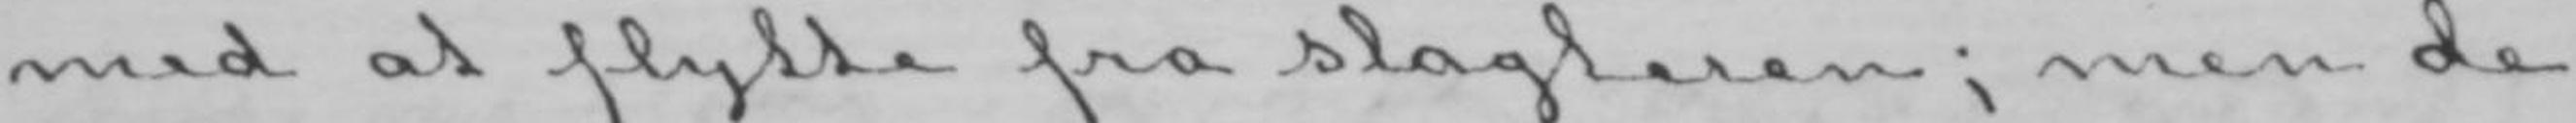med at flytte fra slagteren; men de
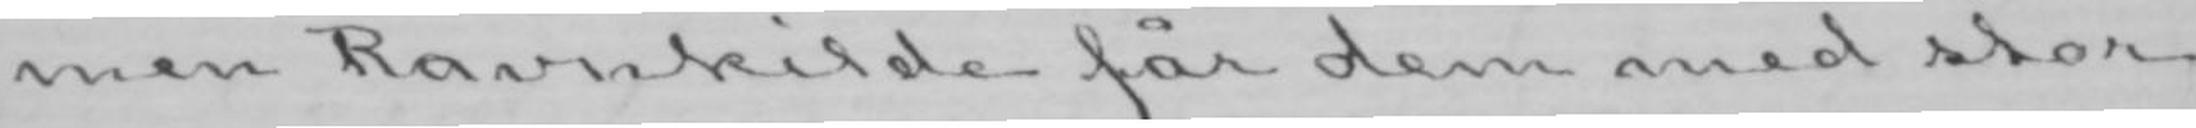men Ravnkilde får dem med stor
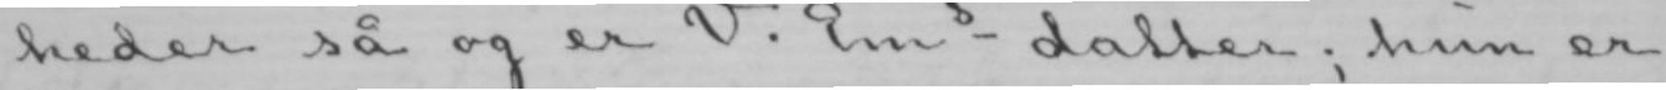heder så og er V. Ems datter; hun er
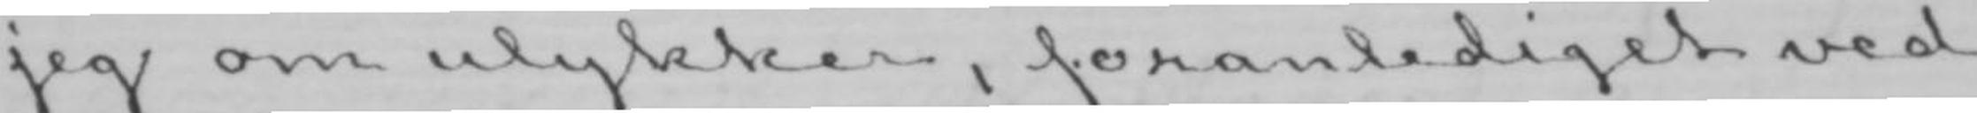jeg om ulykker, foranlediget ved
